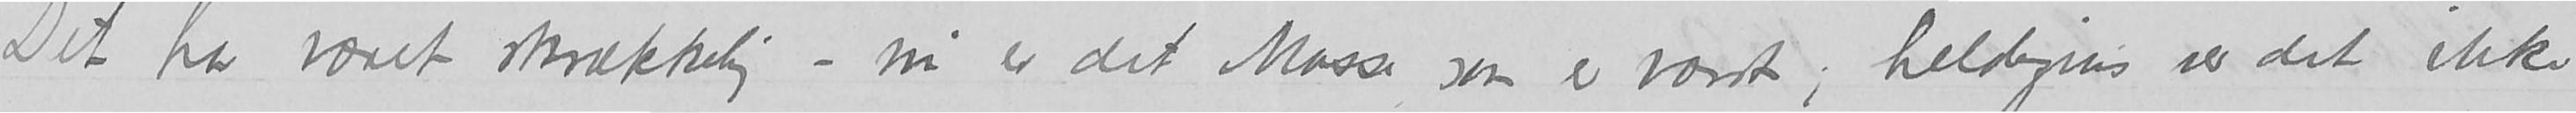Det har været skrækkelig – nu er det Mosse som er værst; heldigvis ser det ikke
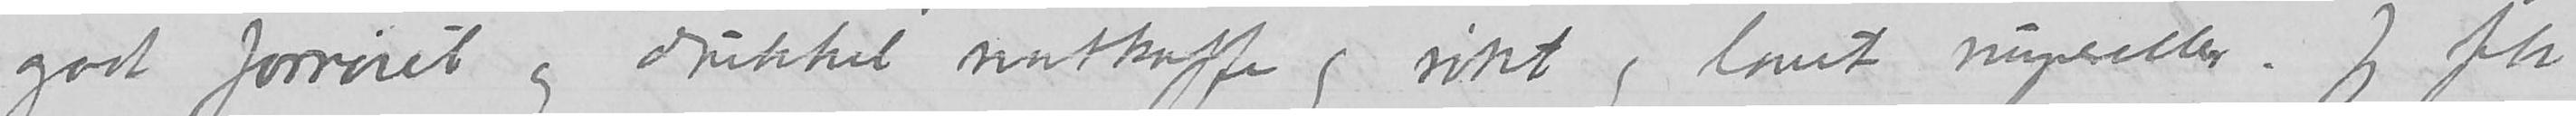godt fornøiet og drukket natkaffe og røkt og lavet nupereller. Jeg fik
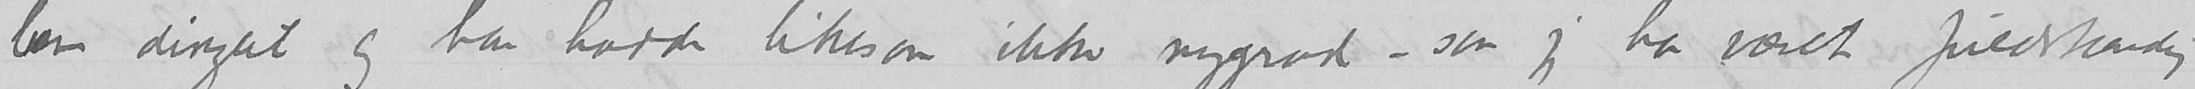ben dinglet og han hadde likesom ikke rygrad – saa jeg har været fuldstendig
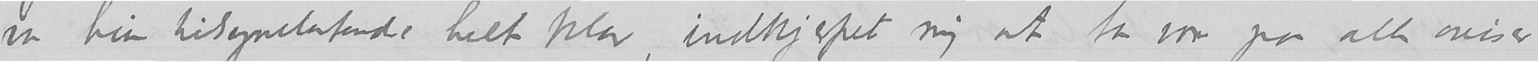var hun tilsynelatende helt klar, indskjerpet mig at ta vare paa alle aviser
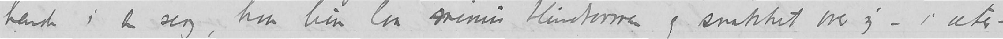hende i en seng, hvor hun laa minus blindtarmen og snakket over sig– i <ute-
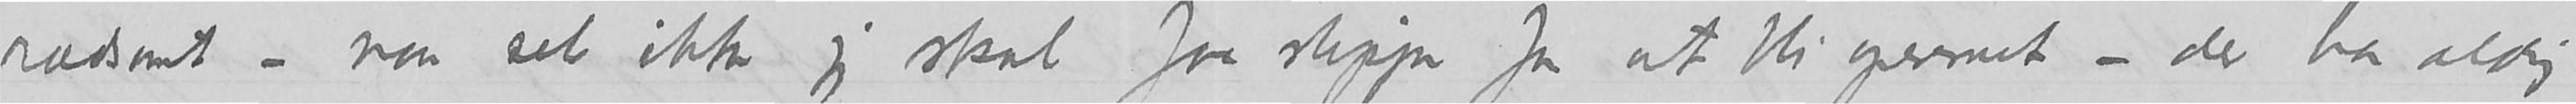rædsomt – naar selv ikke jeg skal faa slippe for at bli opereret - der har aldrig
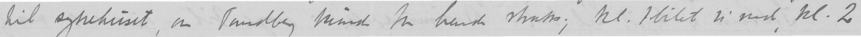til sykehuset, om Tandberg kunde ta hende straks; kl. 7 bilet vi ned, kl. 2
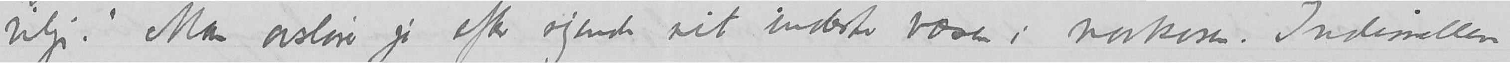vilje.» Man avslører jo efter sigende sit inderste væsen i narkosen. Indiemellem
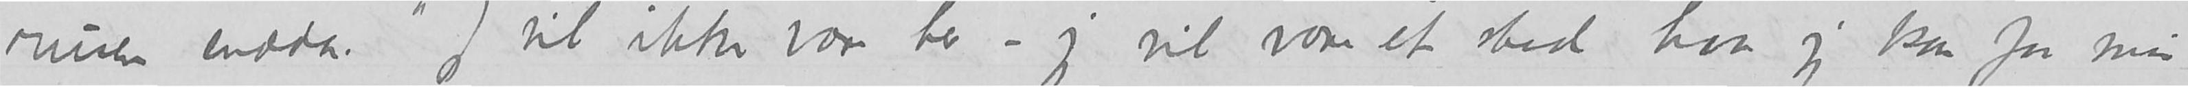rusen> endda.  «Jeg vil ikke være her – jeg vil være et sted hvor jeg kan faa min
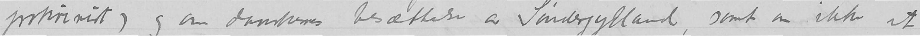prokurist) og om danskenes besættelse av Sønderjylland, samt om ikke at
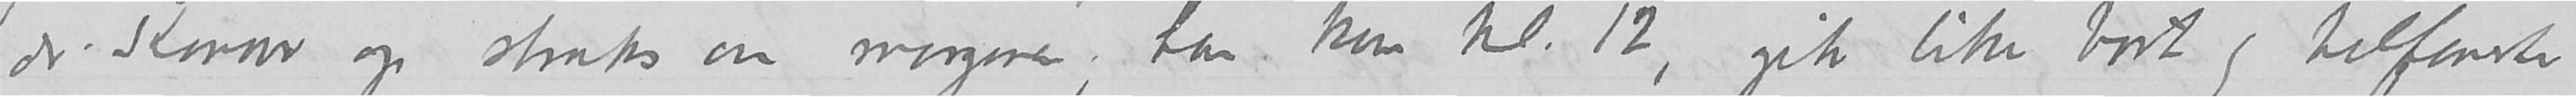dr. Konow op straks om morgenen;han kom kl. 12, gik like bort og telefonerte
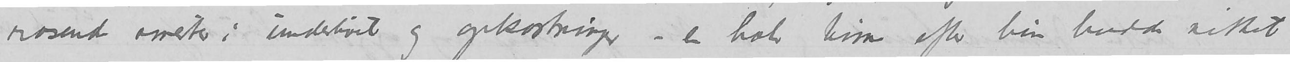rasende smerter i underlivet og opkastninger – en halv time efter hun hadde sittet
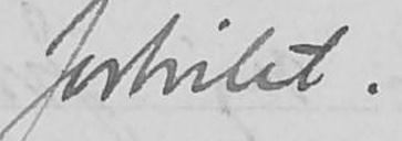fortvilet.
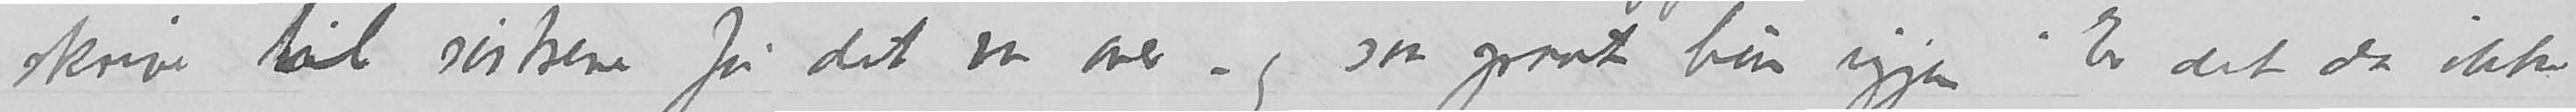skrive til søstrene før det vaer over – og saa graat hun igjen. «Er det da ikke
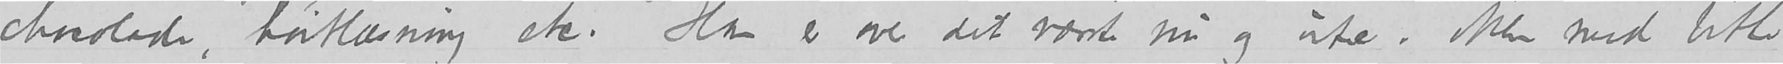chokolade, høitlæsning etc.  Han er over det værste nu og ute. Men med bitte
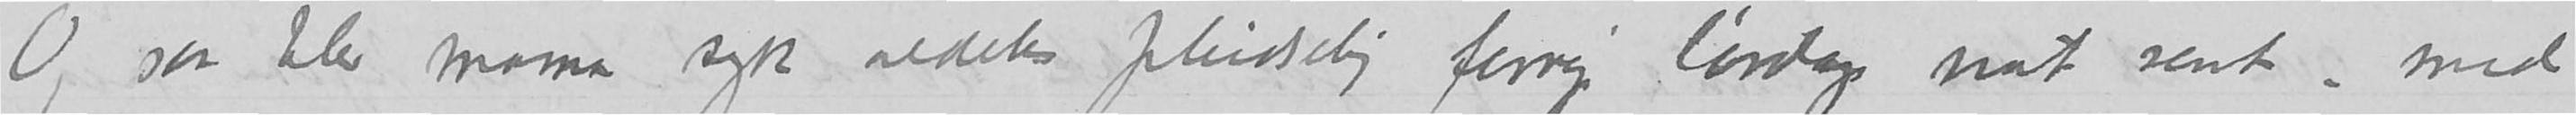Og saa ble mama syk aldeles pludselig forrige lørdags nat sent, – med
